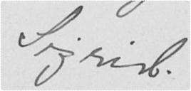Sigrid
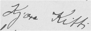Kjære Kitti
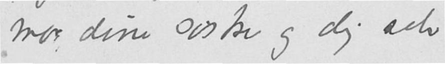mor dine søstre og dig selv
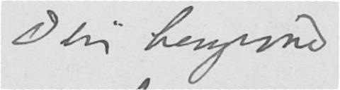Din hengivne
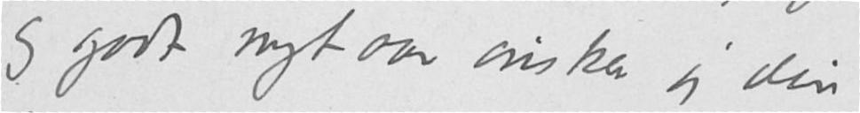og godt nytaar ønsker jeg din
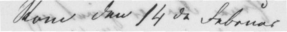Rom den 14de Februar
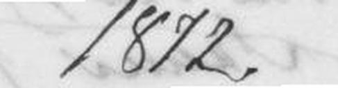1872.
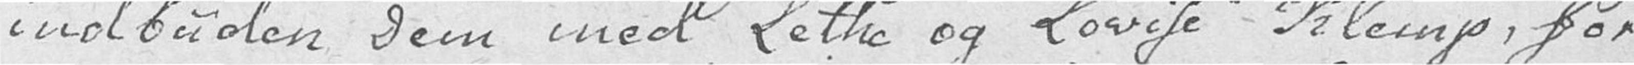indbuden Dem med Lethe og Lovise Klemp, for
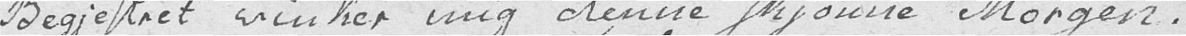Begejstret vinker mig denne skjønne Morgen!
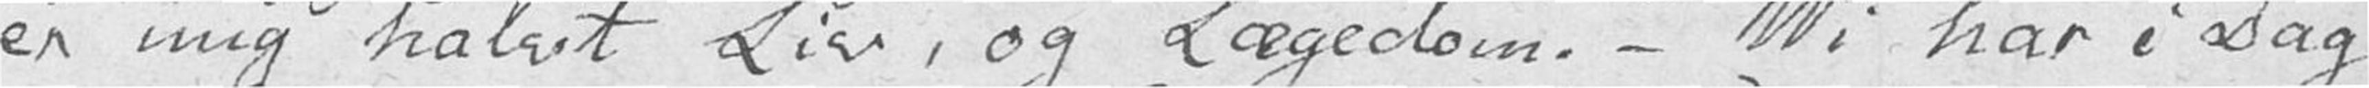er mig halvt Liv, og Lægedom. – Vi har i Dag
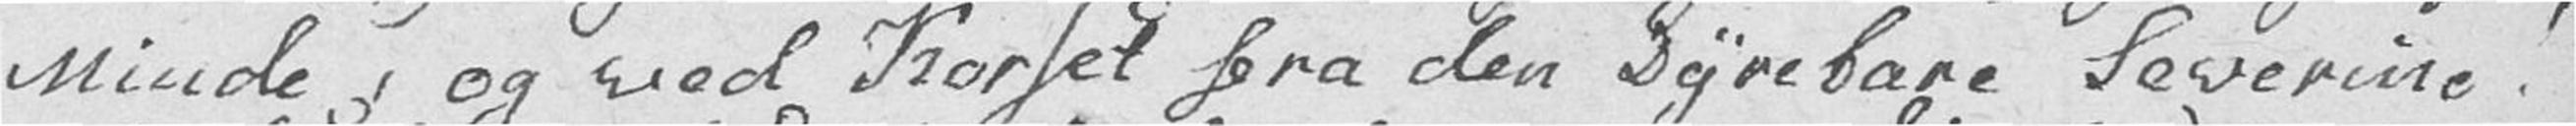Minde, og ved Korset fra den Dyrebare Severine!
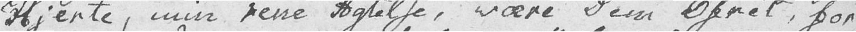Hjerte, min rene Agtelse, være Dem Ofret, for
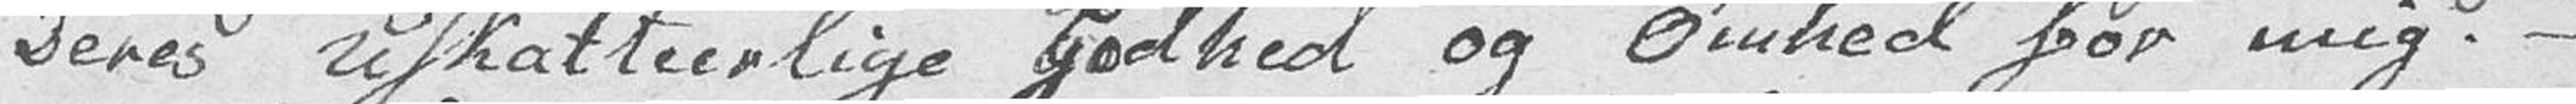Deres uskatteerlige Godhed og Ømhed for mig. –
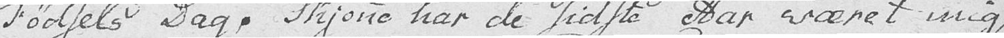Fødsels Dag. Skjønne har de sidste Aar været mig,
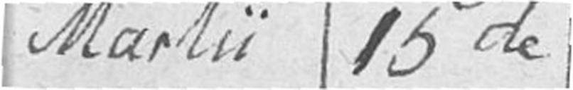Martii 15de
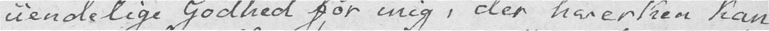uendelige Godhed for mig, der hverken kan
